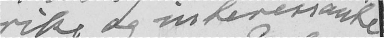rike og interessante
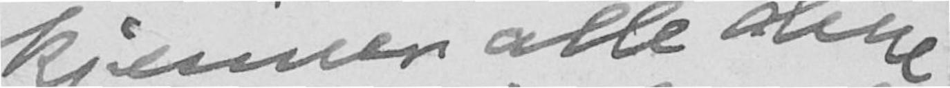kjenner alle dine
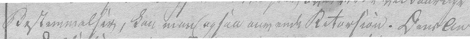Bestemmelser, kan man ogsaa anvende Retorsion. Dens An-
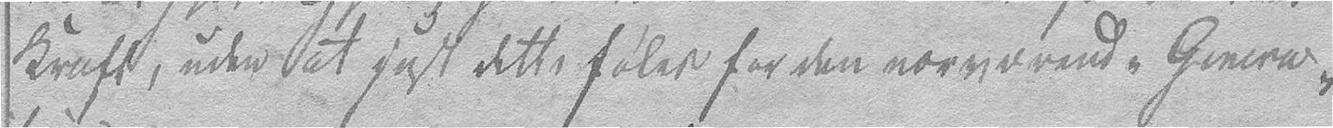Kraft, uden at just dette føles for den nærværende Genera-
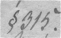§ 315.
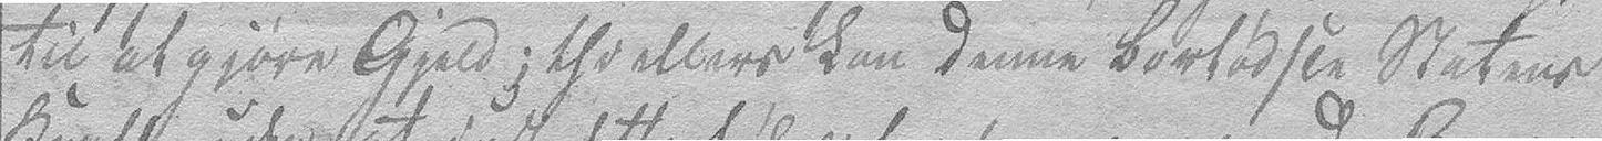til at gjøre Gjeld; thi ellers kan denne bortødsle Statens
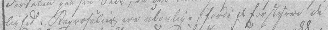lighed. Repræsalier ere ulovlige fordi de forstyrre de
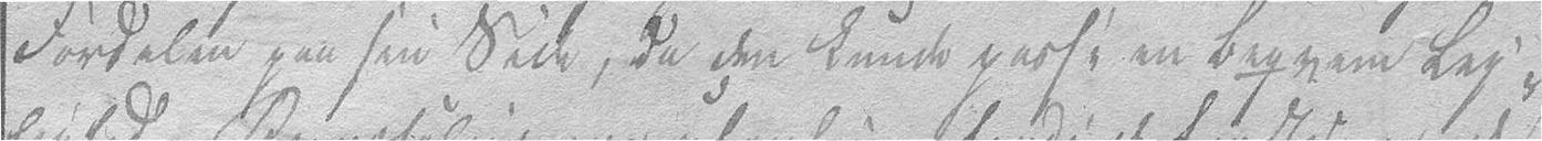Fordelen paa sin Side, da den kunde passe en beqvem Lej-
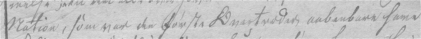Nation, som var den første Overtræder aabenbare have
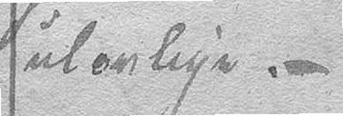ulovlige.–
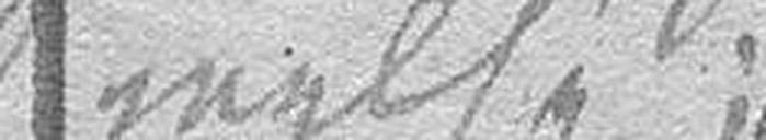melse;
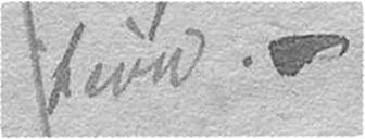tion. –
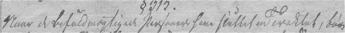Naar de befuldmægtigede Personer have sluttet en Traktat, bør
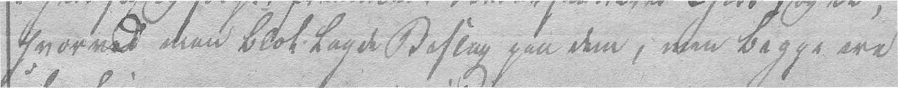hvorved man blot lagde Beslag paa dem, men begge ere
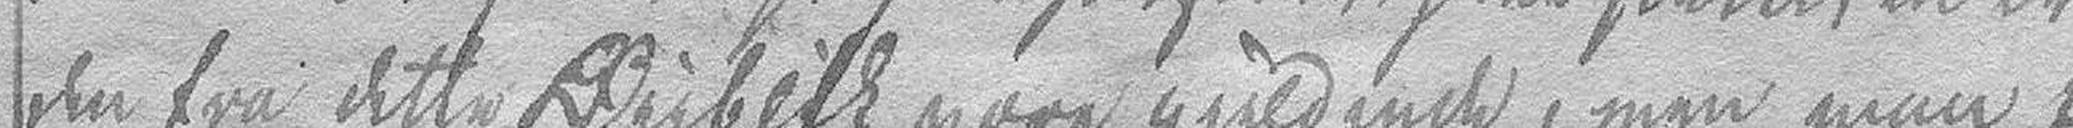den fra dette Øieblik være gjeldende, men man
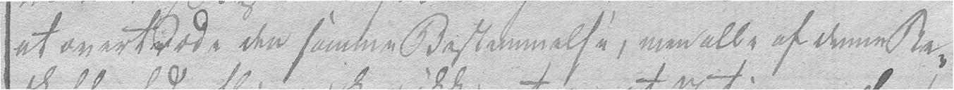at overtræde den samme Bestemmelse, men alle af denne Be-
